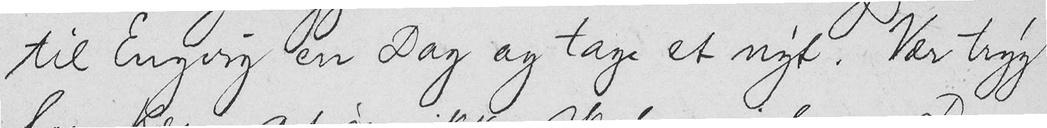til Engerig en Dag og tage et nyt. Vær tryg
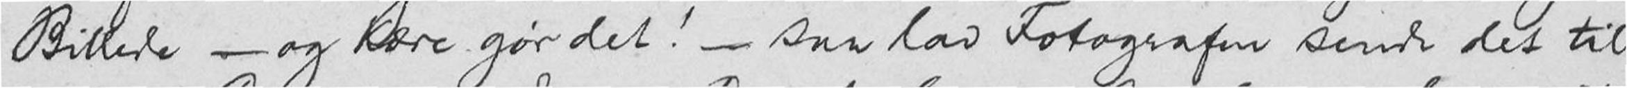Billede - og Kære gør det! - saa lad Fotografen sende det til
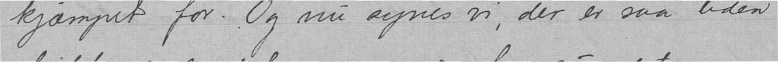kjæmpet for. Og nu synes vi, der er saa liden
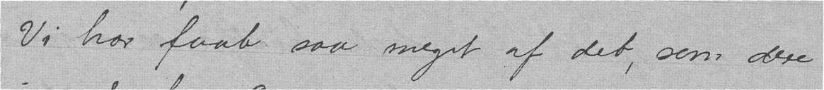Vi har faat saa meget af det, som dere
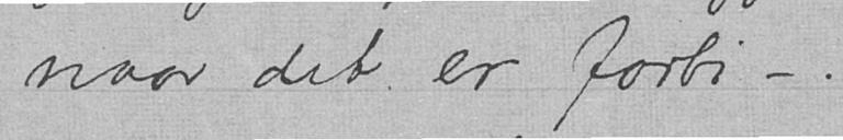naar det er forbi -.
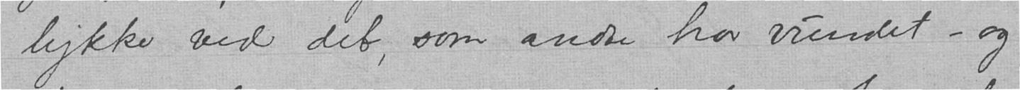lykke ved det, som andre har vundet - og
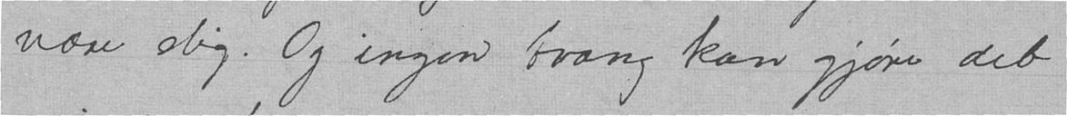være slig. Og ingen tvang kan gjøre det
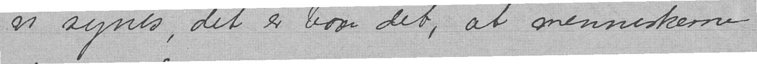vi synes, det er bare det, at menneskerne
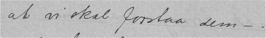at vi skal forstaa dem - .
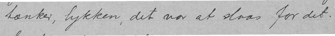tænker, lykken, det var at slaas for det.
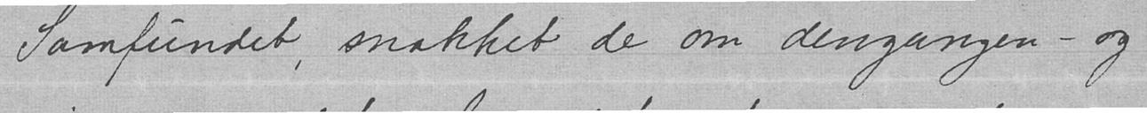Samfundet, snakket de om dengangen - og
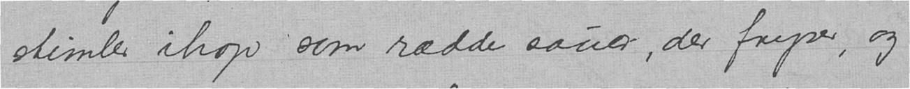stimler ihop som rædde sauer, der fryser, og
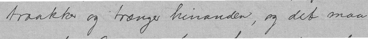traakker og trænger hinanden, og det maa
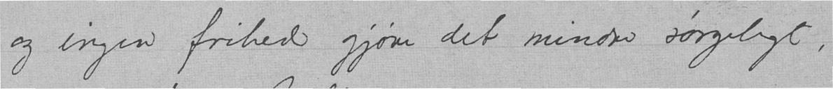og ingen frihed gjøre det mindre sørgeligt,
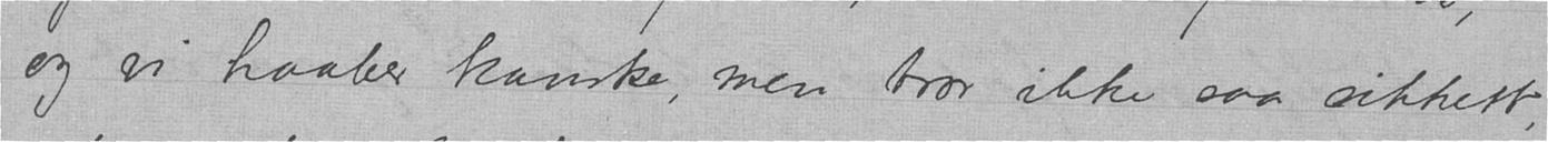og vi haaber kanske, men tror ikke saa sikkert,
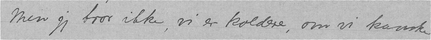Men jeg tror ikke, vi er koldere, om vi kanske
